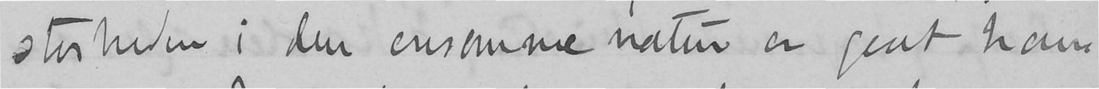storheden i den ensomme natur er gaat ham
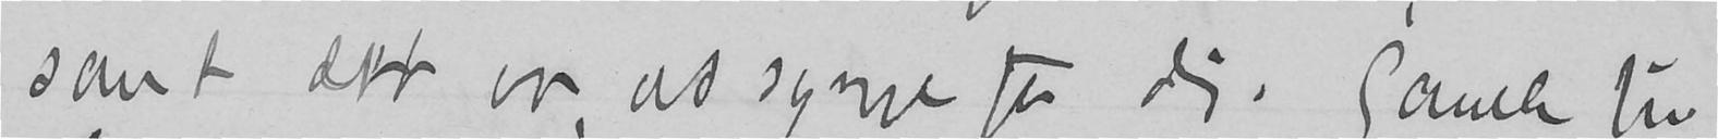somt det var, at synge for dig. Gamle Liv
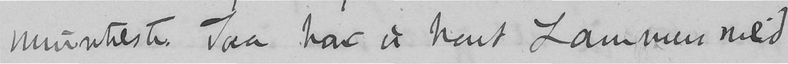muntreste. Saa har vi havt Lammers med
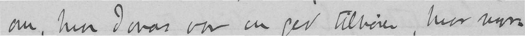om, hvor Jonas var en god tilhører, hvor mor-
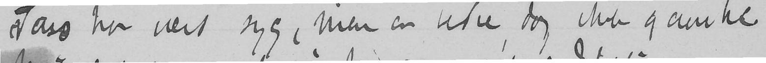Sars har vært syg, men er bedre, dog ikke ganske.
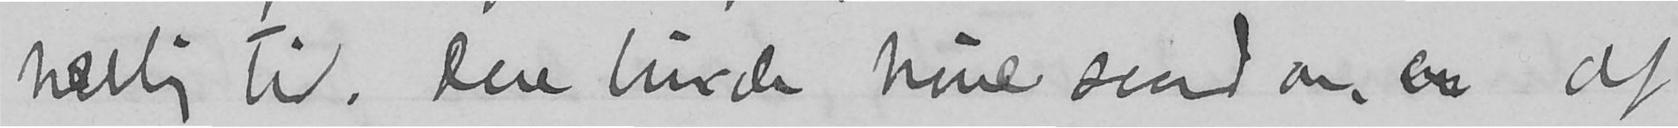herlig tid. Dere burde høre saadan en af
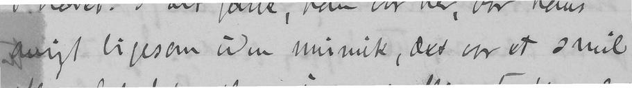ansigt ligesom uden mimik, det var et smil
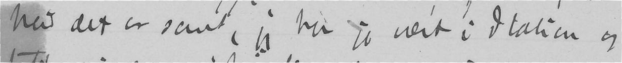Nei det er sant, jeg har jo vært i Italien og
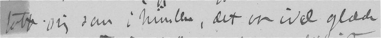borte slig som i München, det er idel glæde
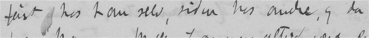først hos ham selv, siden hos andre, og da
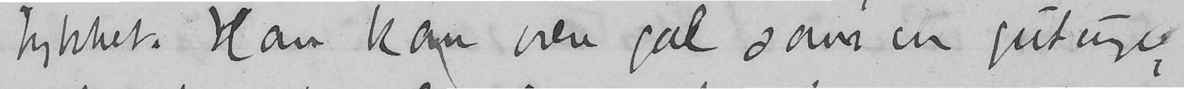trykket. Han kan være gal som en gutunge,
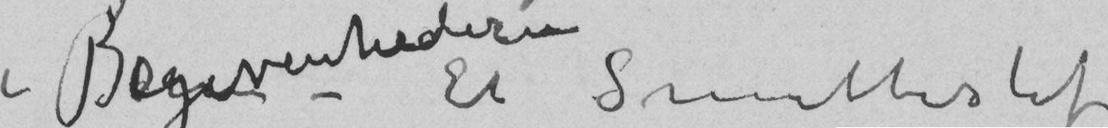i Begivenhederne – Et Smittestof
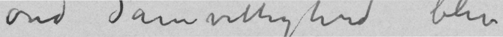ond Samvittighet blev
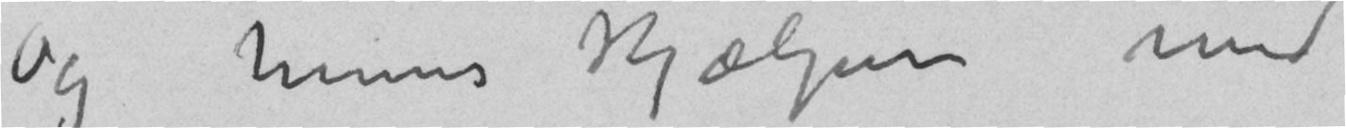Og hennes Hjælpere med
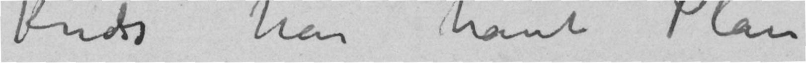Kreds har havt Plan
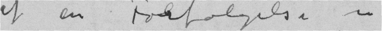af en Forfølgelse i
